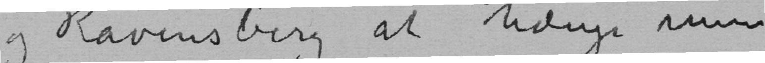og Ravensberg at hænge mine
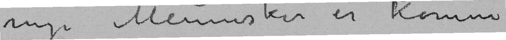nye Mennesker er komne
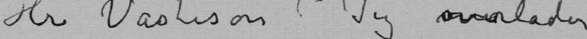Hr Vasteson! Jeg overlader
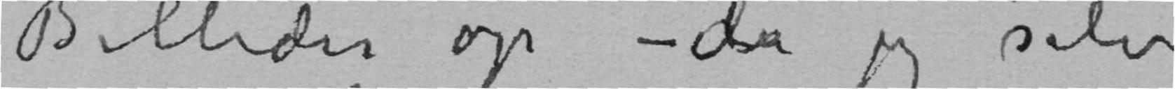Billeder op – da jeg selv
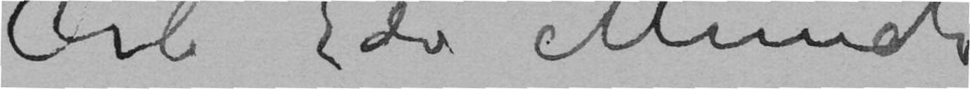Ærb Edv Munch
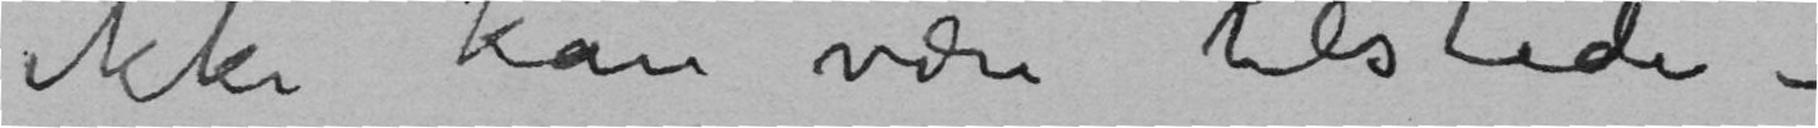ikke kan være tilstede –
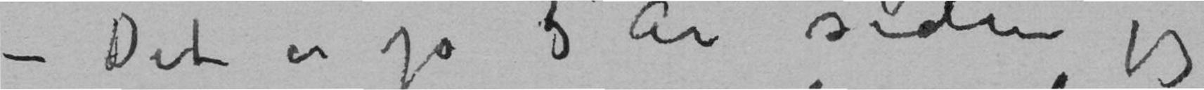– Det er jo 5 År siden jeg
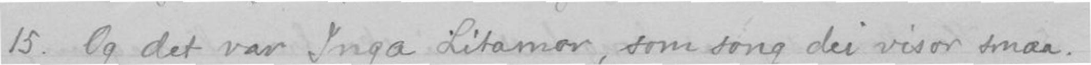15. Og det var Inga Litamor, som song dei visor smaa.
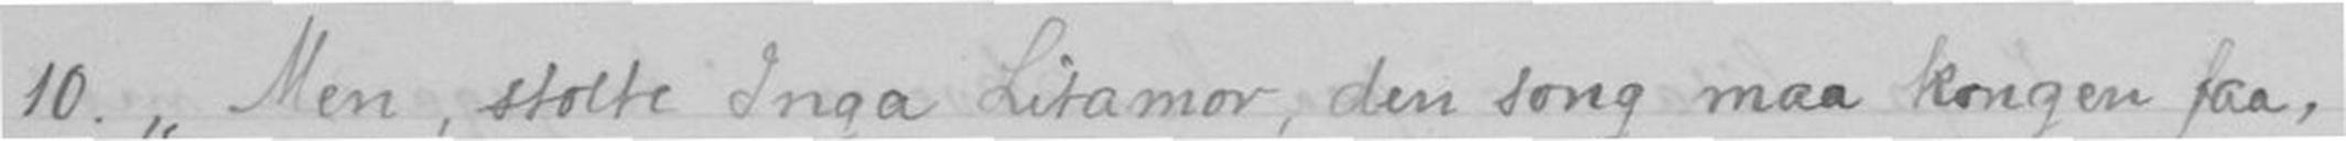10. Men, stolte Inga Litamor, den song maa kongen faa,
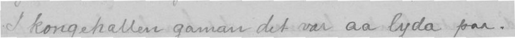I kongehallen gaman det var aa lyda paa.
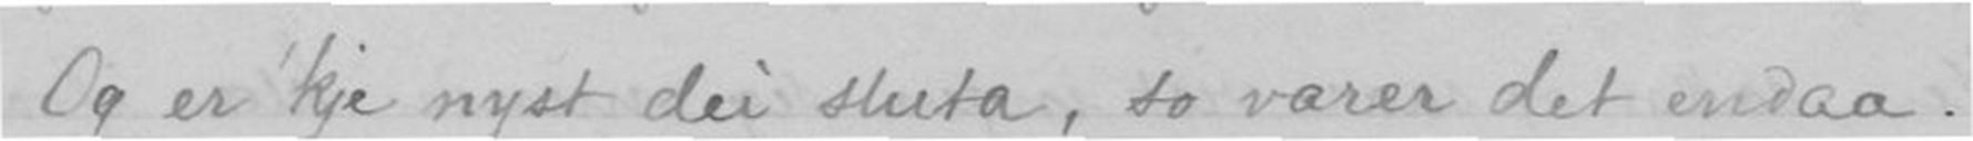Og er 'kje nyst dei sluta, so varer det endaa
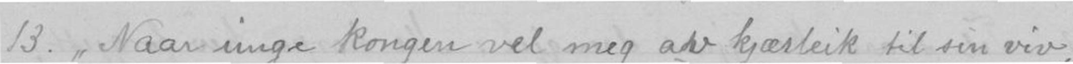13. Naar unge kongen vel meg av kjærleik til sin viv,
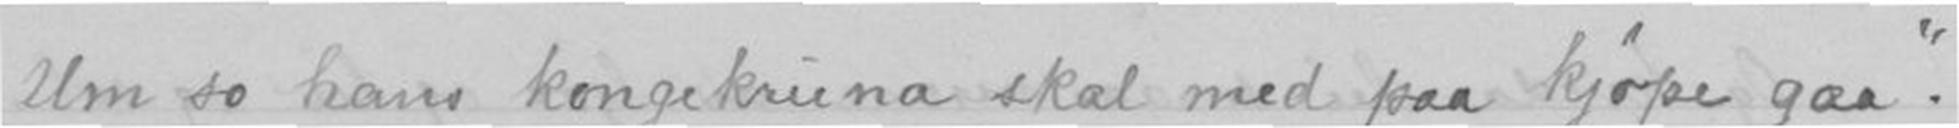Um so hans kongekruna skal med paa kjøbet gaa.
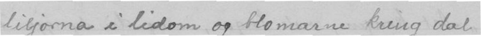liljorna i lidom og blomarne kring dal
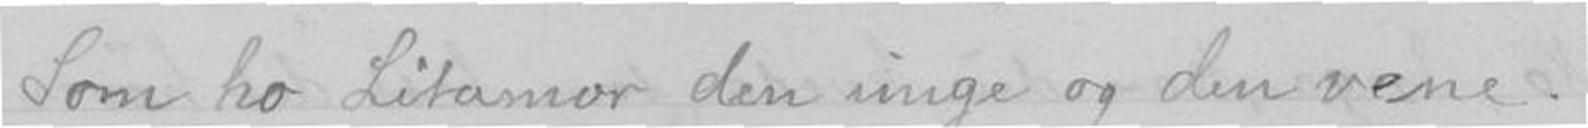Som ho Litamor den unga og den vene.
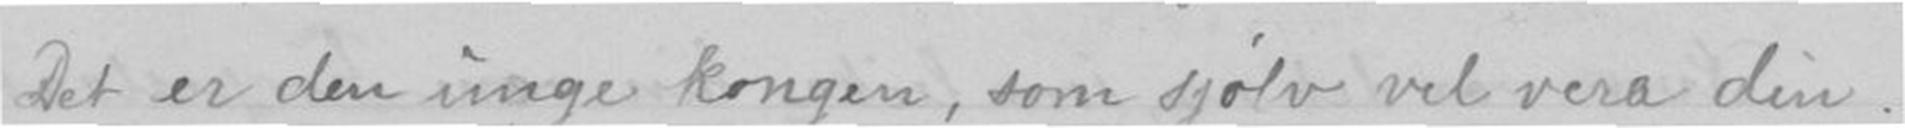Det er den unge kongen, som sjølv vil vera din.
